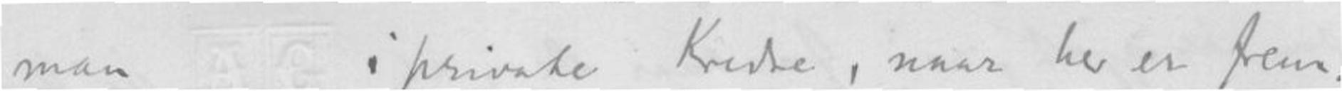man i privade Kretser, naar her er frem-
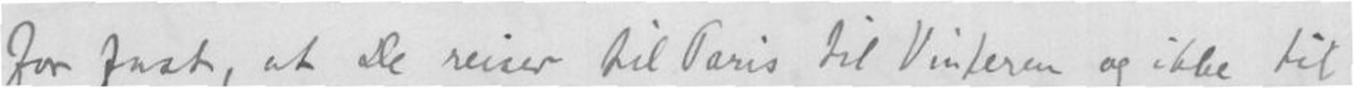for fort, at De reiser til Paris til Vinteren og ikke til
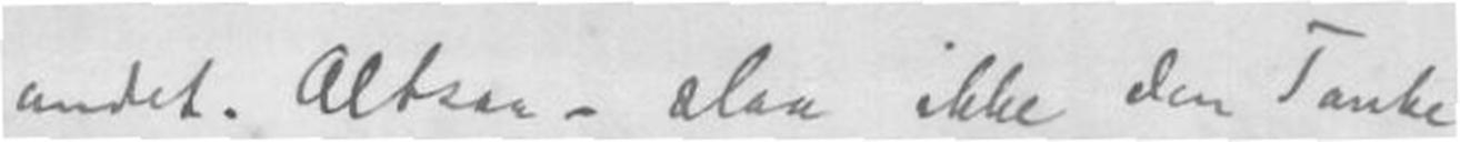andet. Altsaa, slaa ikke den Tanke
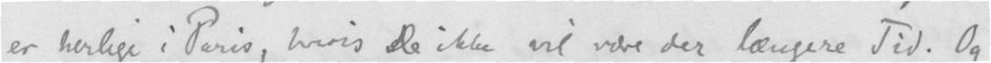er herlige i Paris, hvis de ikke vil være der længere Tid Og
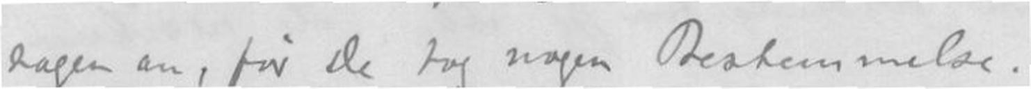sagen an, før De tar nogen Bestemmelse.
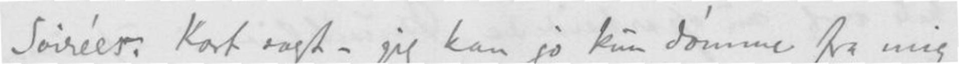Soiréer. Kort sagt, jeg kan jo kun dømme fra mig
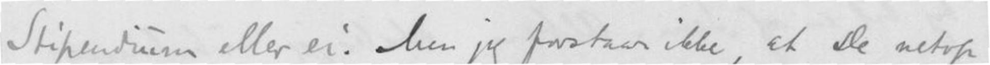Stipendium eller ei. Men jeg forstaar ikke, at De netop
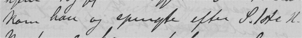kom han og spurgte efter S. 1ste H.
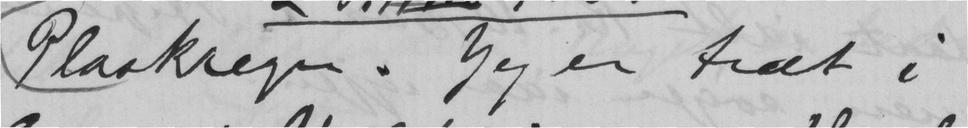Plaskregn. Jeg er træt i
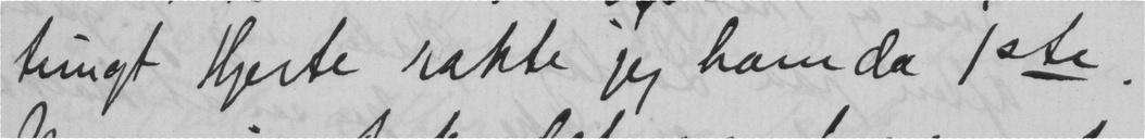tungt Hjerte rakte jeg ham da 1ste.
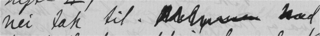nei tak til.  Med
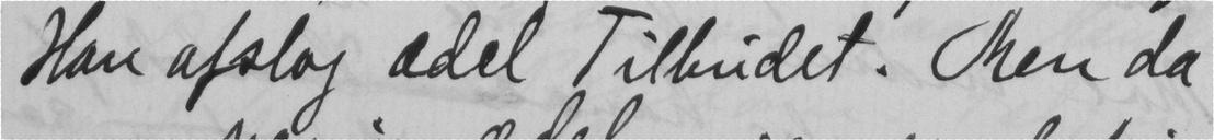Han afslog ædel Tilbudet. Men da
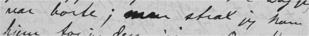var bort; men strax jeg kom
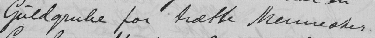Guldgrube for trætte mennesker.
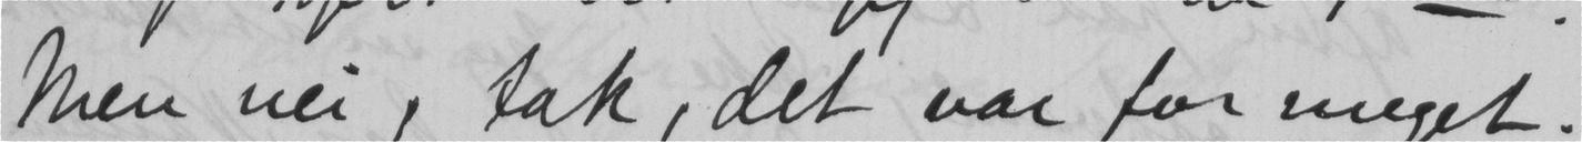Men nei, tak, det var for meget.
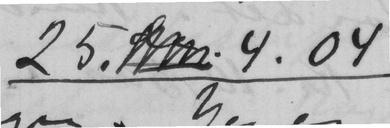25.. 4.04
